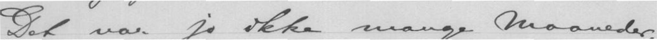Det var jo ikke mangen Maaneder,
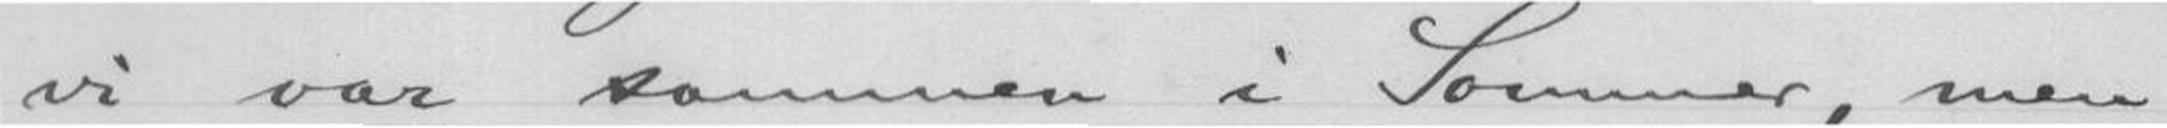vi var sammeni Sommer, men
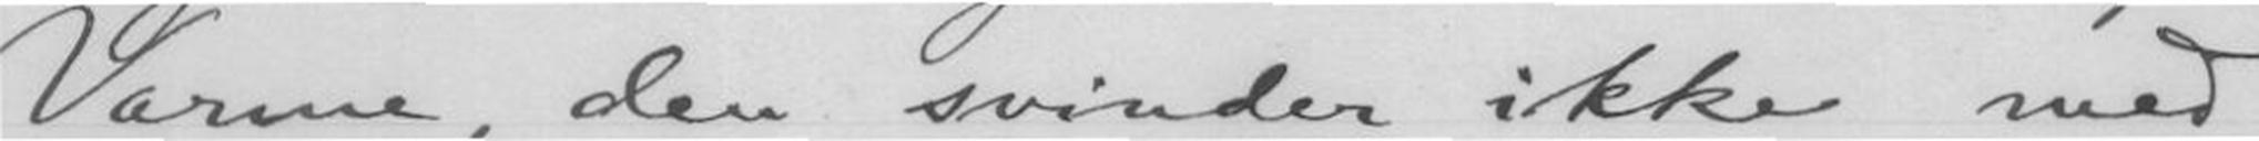Varme, den svinder ikke med
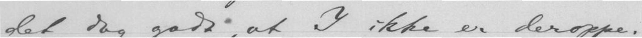det dog godt, at I ikke er deroppe.
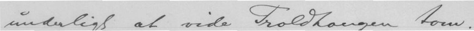underligt at vide Troldhaugen tom;
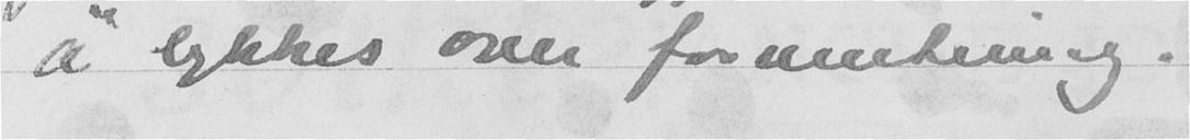å lykkes over forventning.
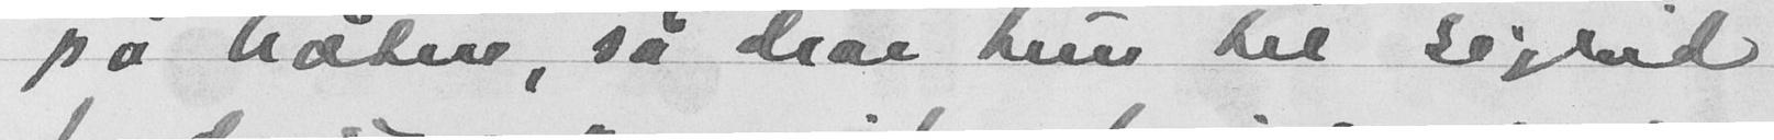på båten, så drar hun til Sigrid
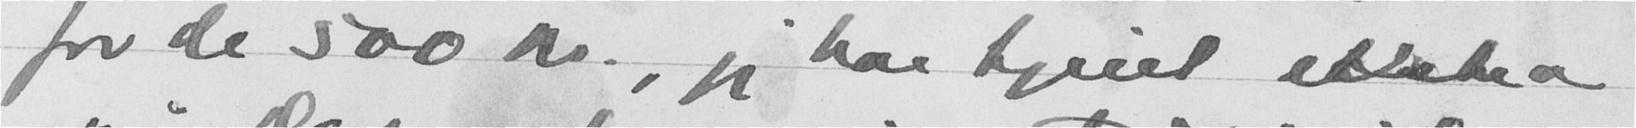for de 500 kr., jeg har tjent ellabra
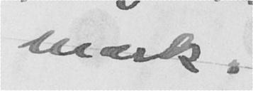mark.
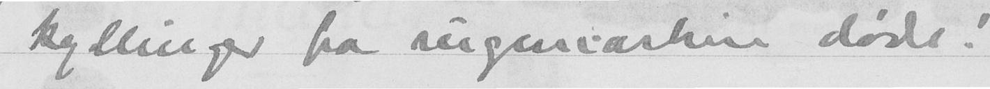kyllinger fra rugemaskin døde!)
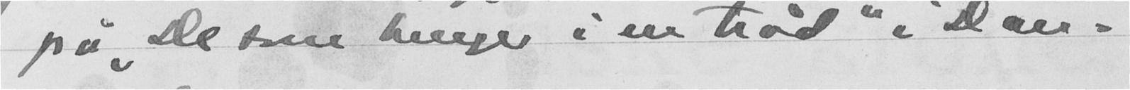på "De som henger i en tråd" i Dan-
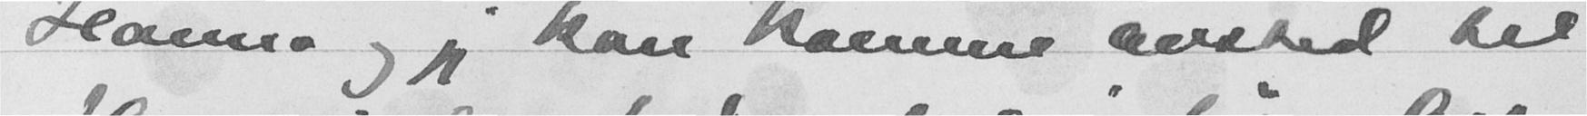Hanna og jeg kan komme avsted til
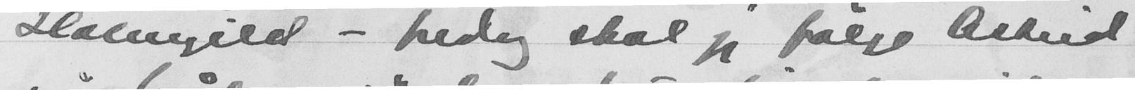Holmgild - fredag skal jeg følge Astrid
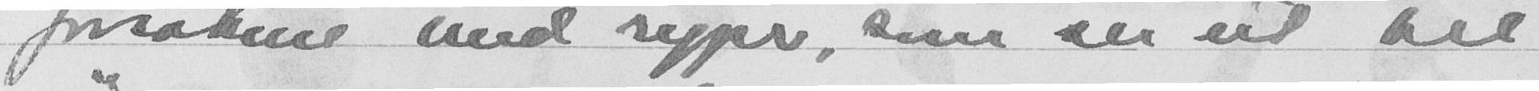forsøkene med ryper, som ser ut til
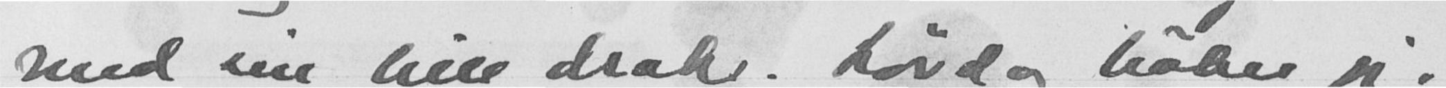med sin lille drake. Lørdag håber jeg.
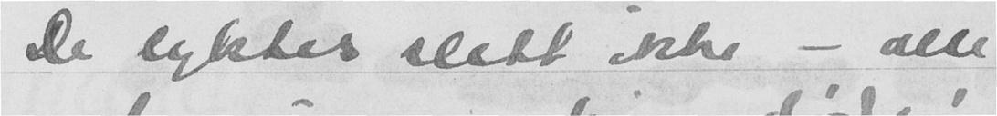(De lyktes slett ikke - alle
